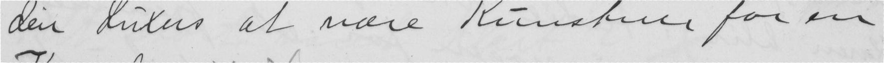den Luxus at være Kunstner for en
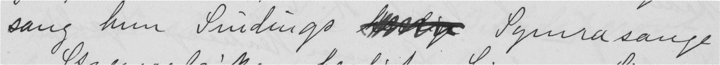sang hun Sindings  Symrasange
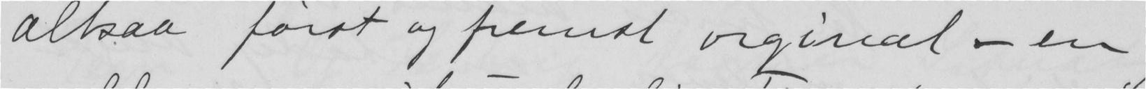altsaa først og fremst orginal - en
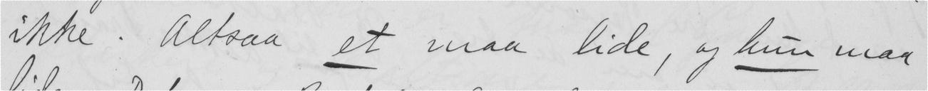ikke. Altsaa et maa lide, og hun maa
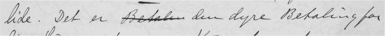lide. Det er  den dyre Betaling for
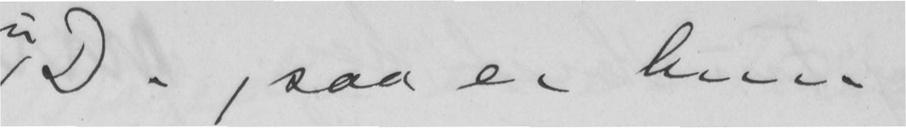D., saa er hun
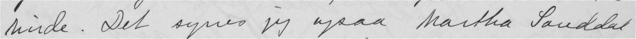rinde. Det synes jeg ogsaa Martha Sanddal
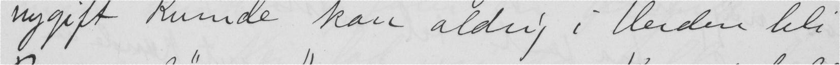nygift Kvinde kan aldrig i Verden bli
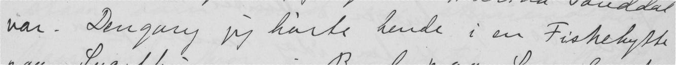var. Dengang jeg hørte hende i en Fiskehytte
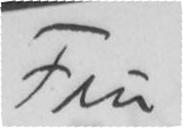Fru
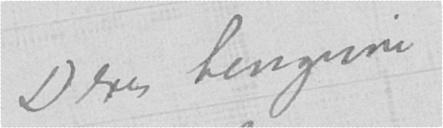Deres hengivne
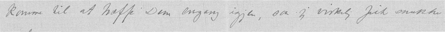komme til at træffe Dem engang igjen, saa jeg virkelig fiik snakke
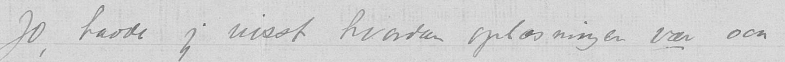Jo, hadde jeg visst hvordan oplæsningen var saa
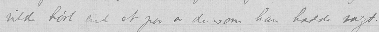vilde hørt end et par av de som han hadde valgt.
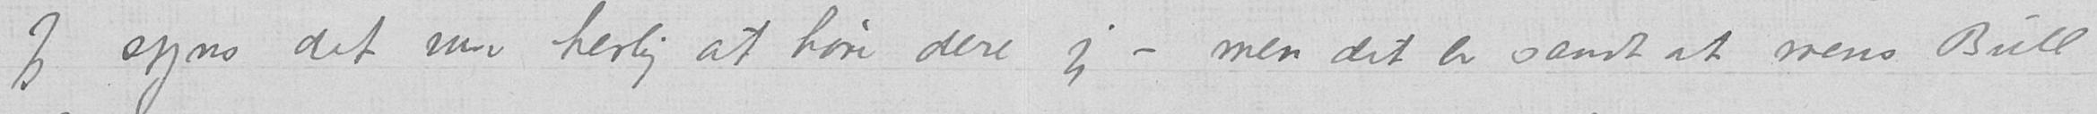Jeg syns det var herlig at høre dere jeg –, men det er sandt at mens Bull
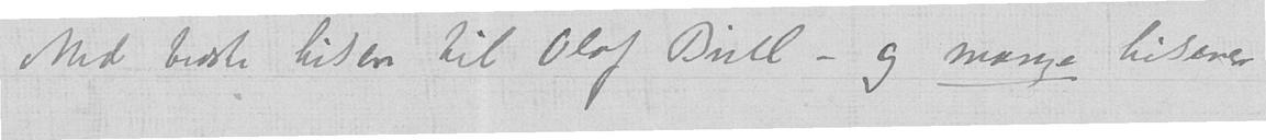Med bedste hilsen til Olaf Bull – og mange hilsener
